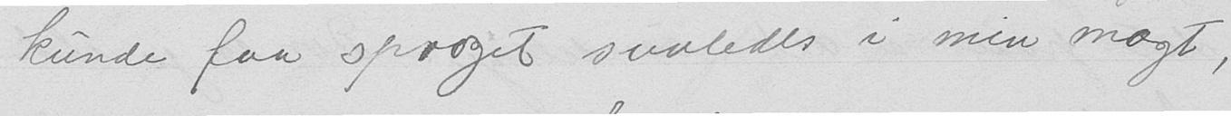kunde faa sproget saaledes i min magt,
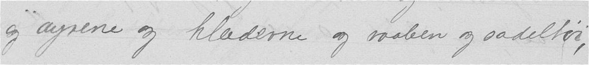og dyrene og klæderne og vaaben og sadeltøi,
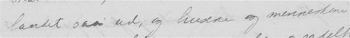landet saa ud, og husene og menneskene
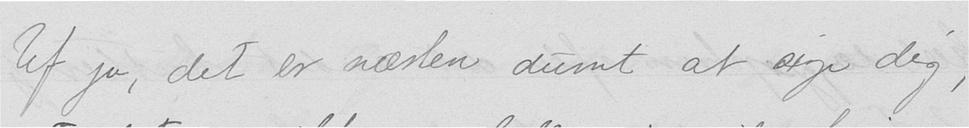Uf ja, det er næsten dumt at sige dig,
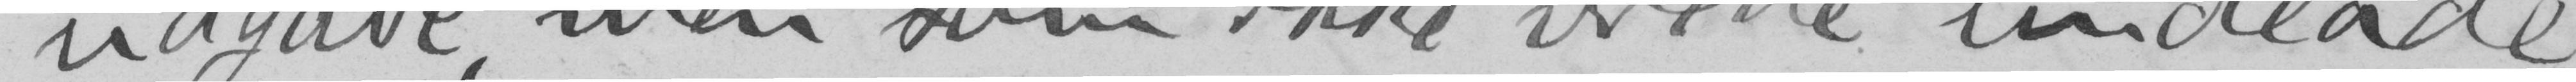udgave, men som ikke vilde undlade
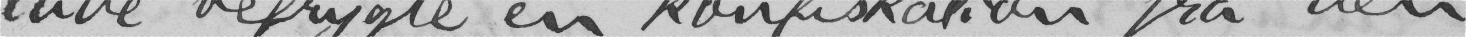lade befrygte en konfiskation fra den
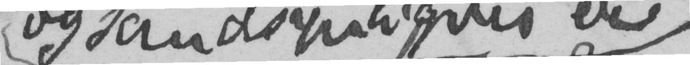og sandsynligvis er
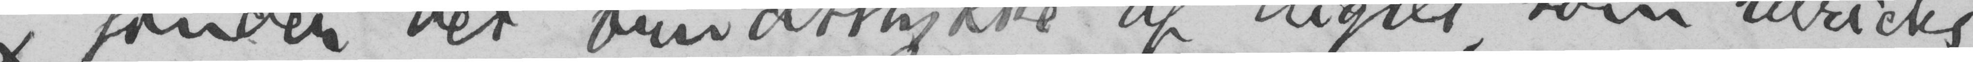finder det brudsstykke af digtet, som Ulrichs
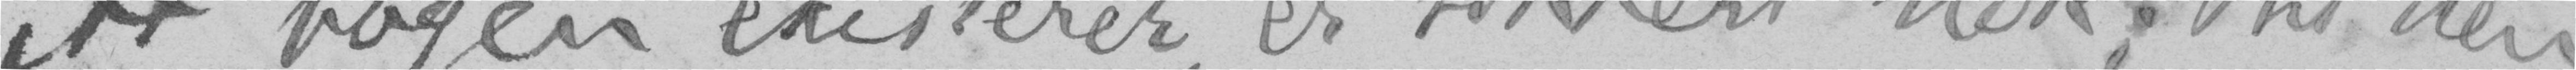At bogen existerer, er sikkert nok; thi den
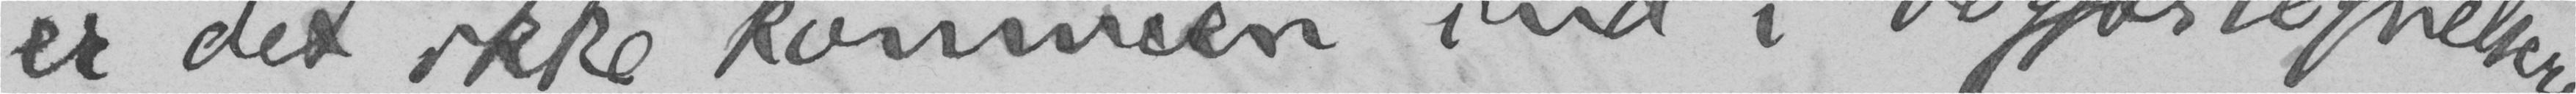er det ikke kommen ind i bogfortegnelserne,
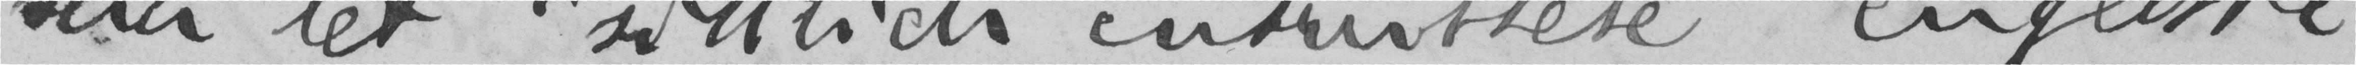saa let «sittlich entrüstete» engelske
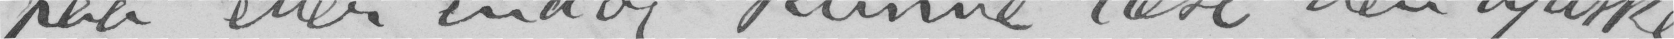paa eller endog kunne læse den tydske
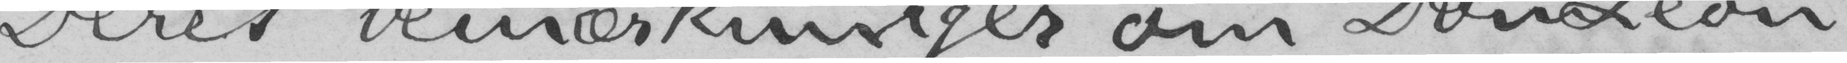Deres bemærkninger om «Don Leon»,
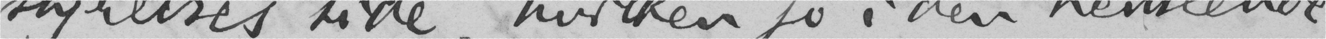styrelses side, hvilken jo i den henseende
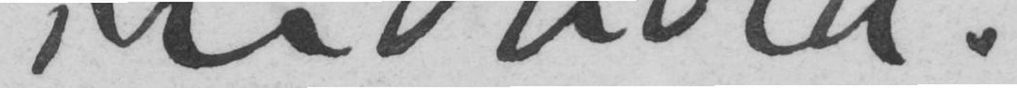indhold.
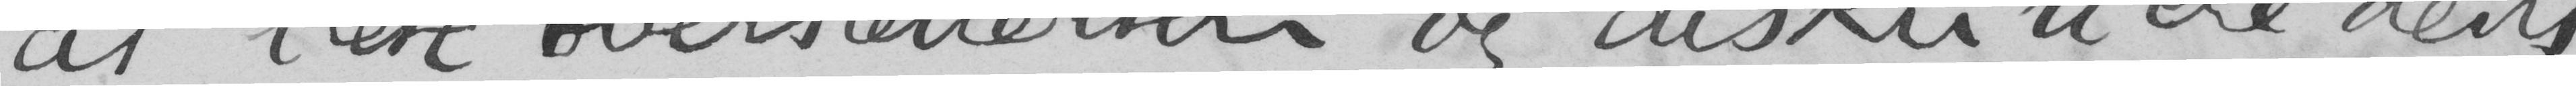at læse oversættelsen og diskuttere dens
